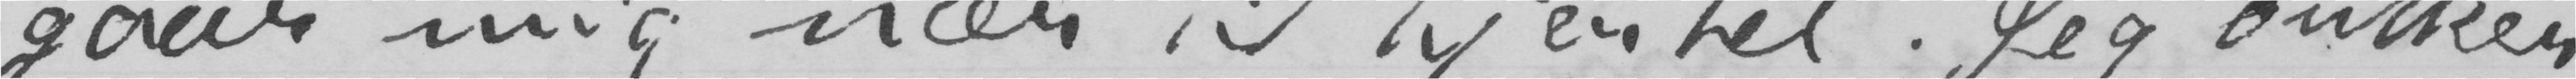gaar mig nær til hjertet. Jeg ønsker
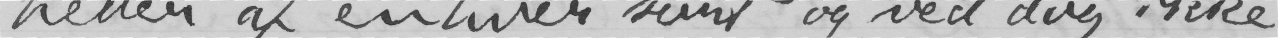heder af enhver sort og ved dog ikke,
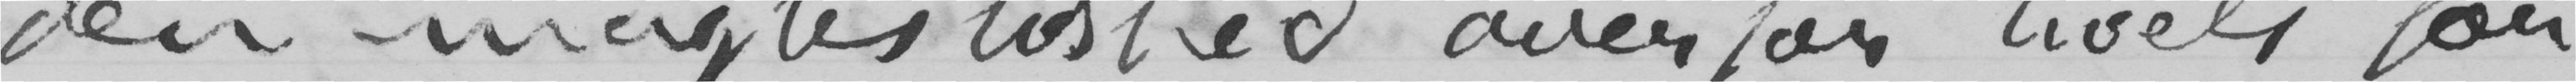den magtesløshed overfor livets for-
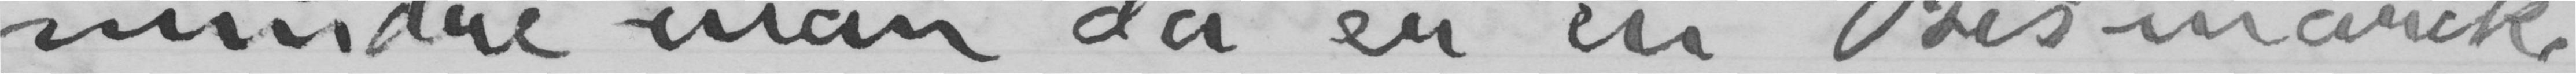mindre man da er en Bismarck
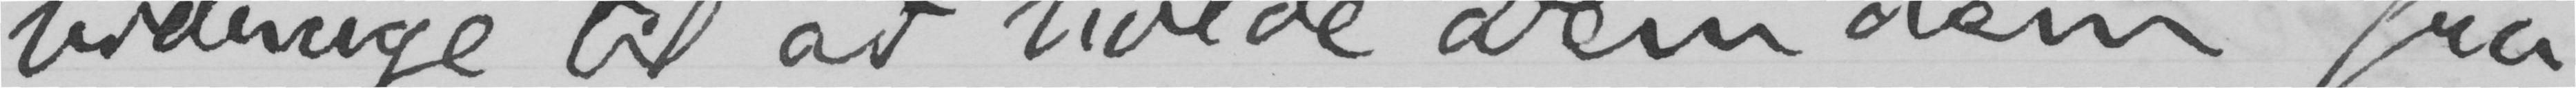bidrage til at holde Dem dem fra
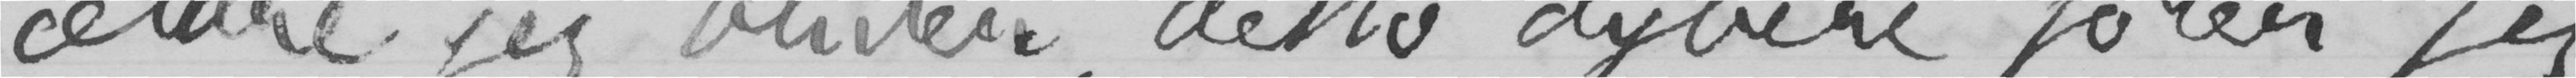ældre jeg bliver, desto dybere føler jeg
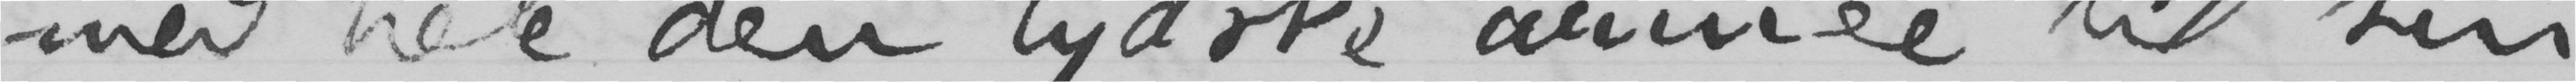med hele den tydske armée til sin
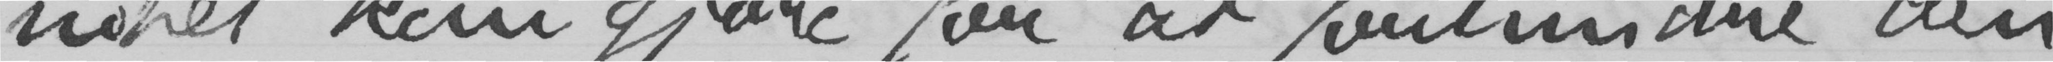intet kan gjøre for at forhindre den.
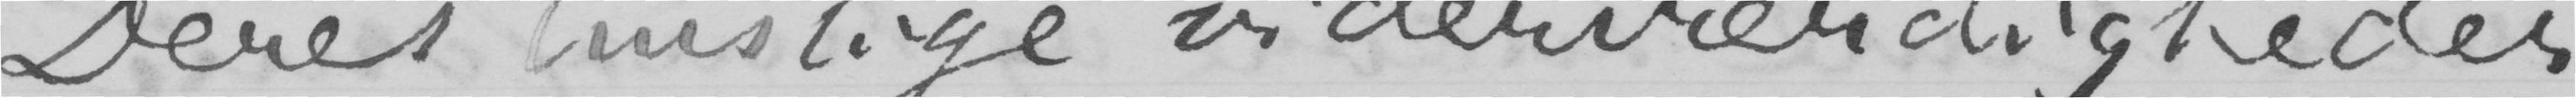Deres huslige viderværdigheder
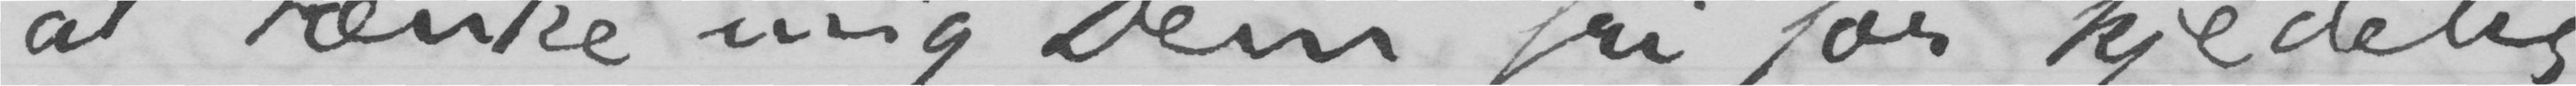at tænke mig Dem fri for kjedelig-
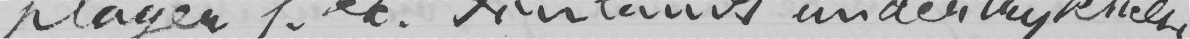plager f.ex. Finlands undertrykkelse
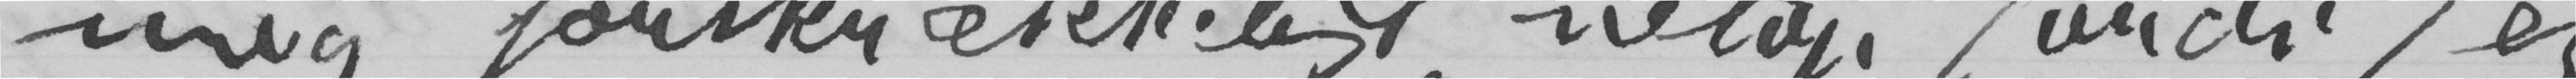mig forskrækkeligt, netop fordi jeg
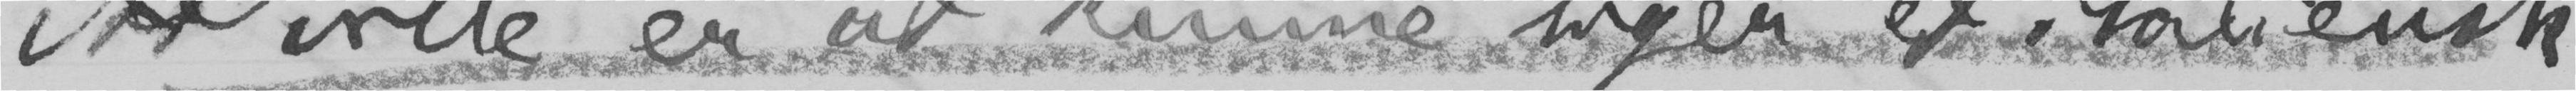At ville er at kunne siger et italiensk
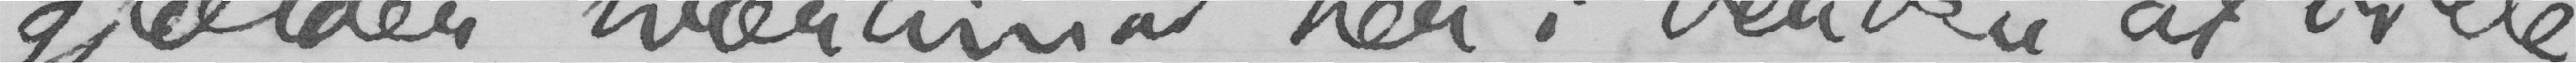gjælder tværtimod her i verden at ville,
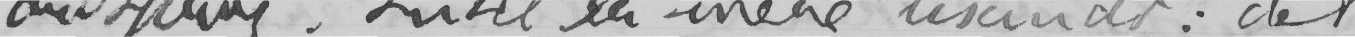ordsprog. Intet er mere usandt: det
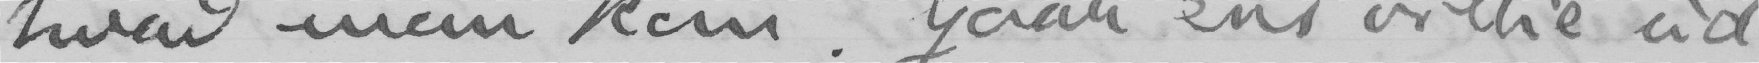hvad man kan. Gaar ens villie ud-
